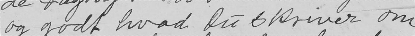og godt hvad Du skriver om
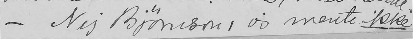- Nej Bjørnson, jeg mente ikke
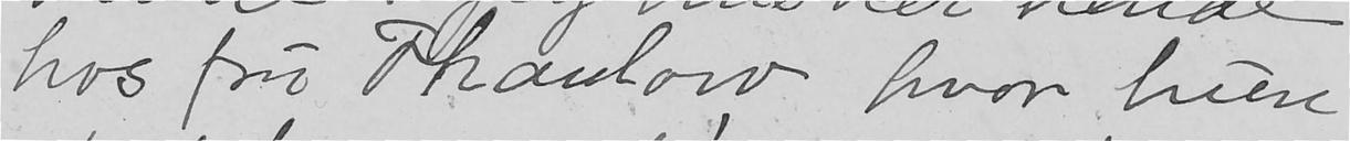hos fru Thaulow, hvor hun
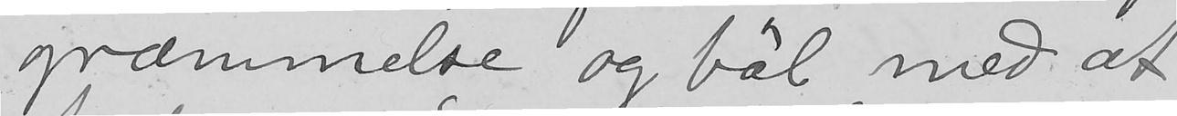græmmelse og bál med at
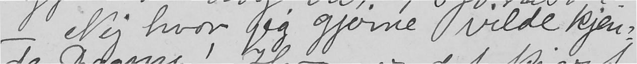- Nej hvor jeg gjerne vilde kjen-
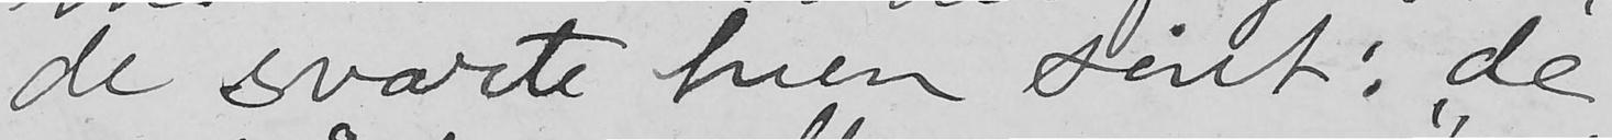de svarte hun sint: "de
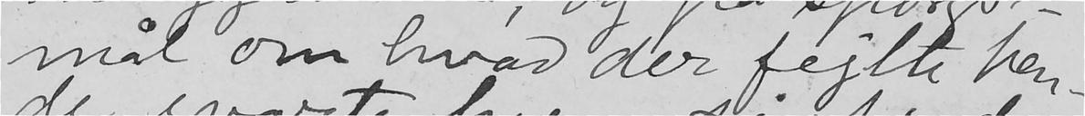mål om hvad der fejlte hen-
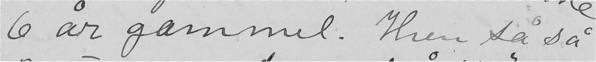6 år gammel. Hun så så
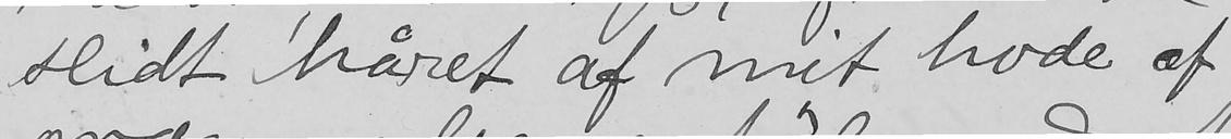slidt håret af mit hode af
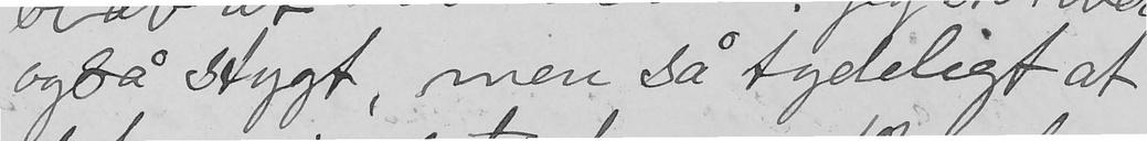også stygt, men så tydeligt at
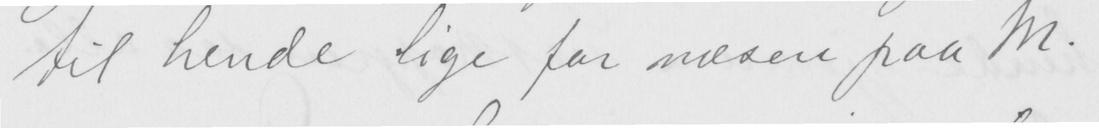til hende lige for næsen paa M.
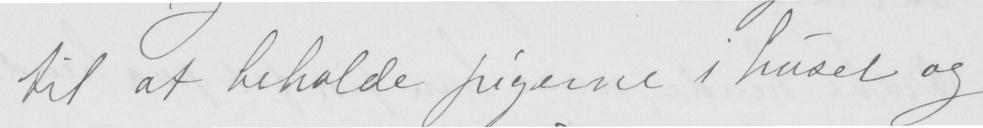til at beholde pigerne i huset og
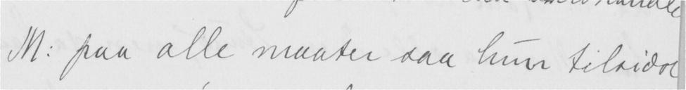M: paa alle maater saa hun tilsidst
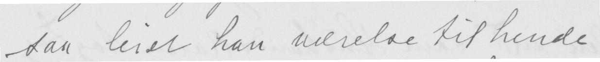saa leiet han værelse til hende
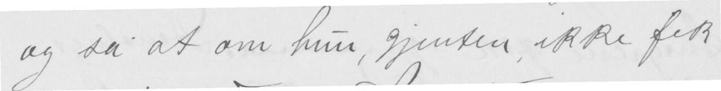og sa at om hun, gjenten, ikke fik
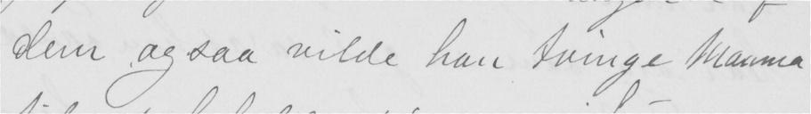dem og saa vilde han tvinge Mamma
